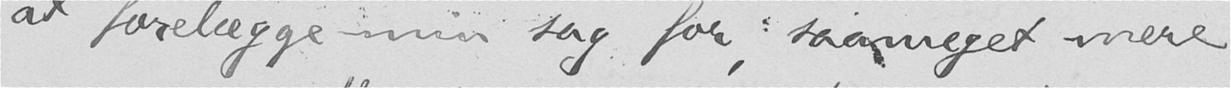at forelægge min sag for, saameget mere
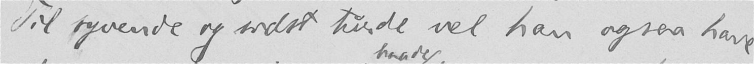Til syvende og sidst turde vel han ogsaa have
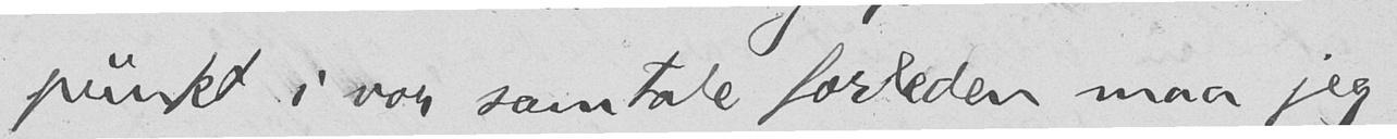punkt i vor samtale forleden maa jeg
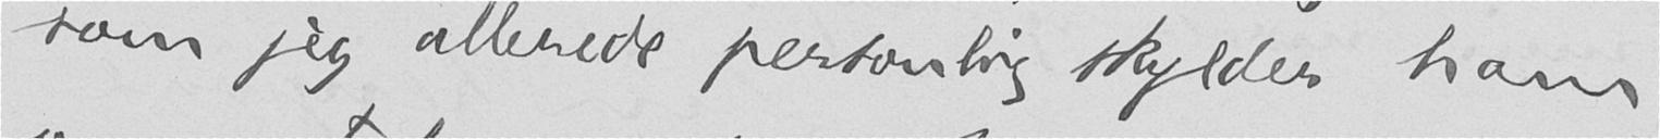som jeg allerede personlig skylder ham
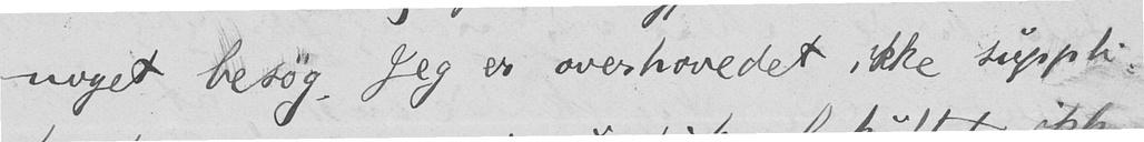noget besøg. Jeg er overhovedet ikke suppli-
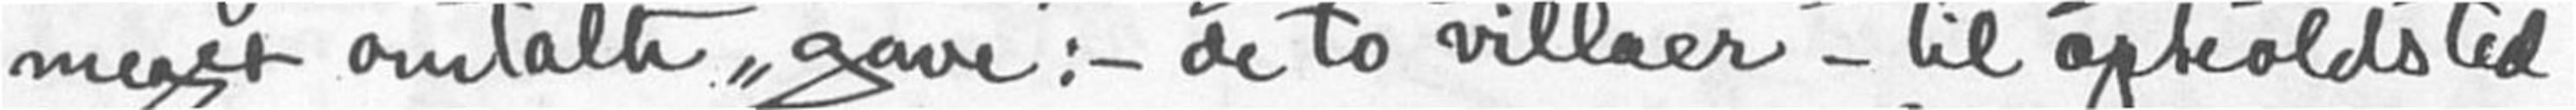meget omtalte "gave" : - de to villaer - til opholdsted
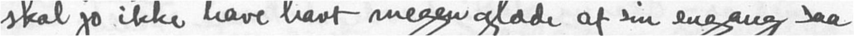skal jo ikke have havt megen glæde af sin engang saa
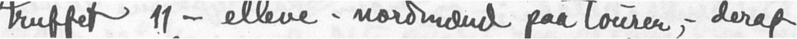truffet 11 - elleve - nordmænd paa touren, - deraf
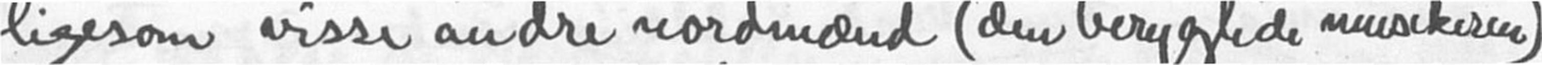ligesom visse andre nordmænd (den berygtede musikeren)
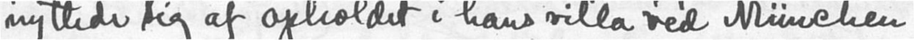nyttede sig af opholdet i hans villa ved München
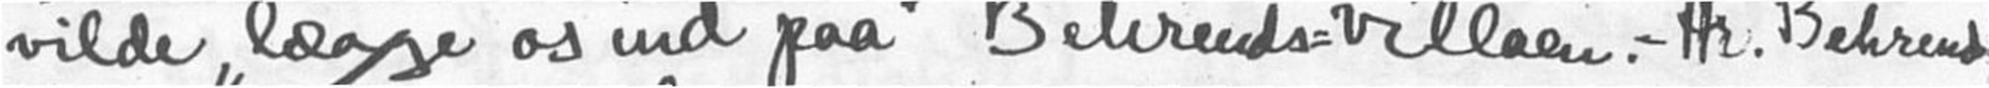vilde "lægge" os ind paa Behrends-villaen. Hr. Behrends
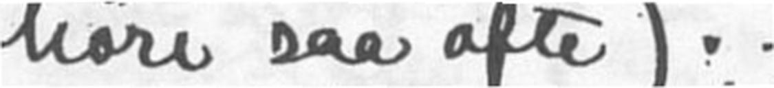høre saa ofte). —
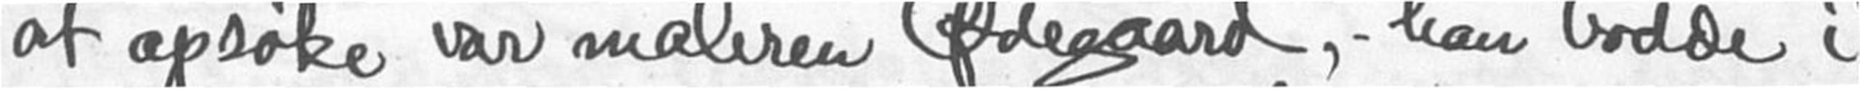at opsøke var maleren Ødegaard, - han bodde i
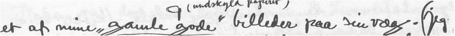et af mine "gamle gode" billeder paa sin væg. (Jeg
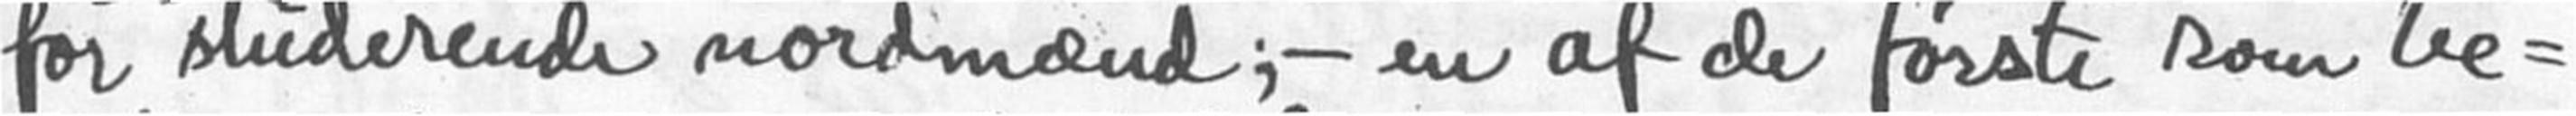for studerende nordmænd ; - en af de forste som be-
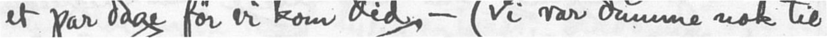et par dage for vi kom did, - (vi var dumme nok til
